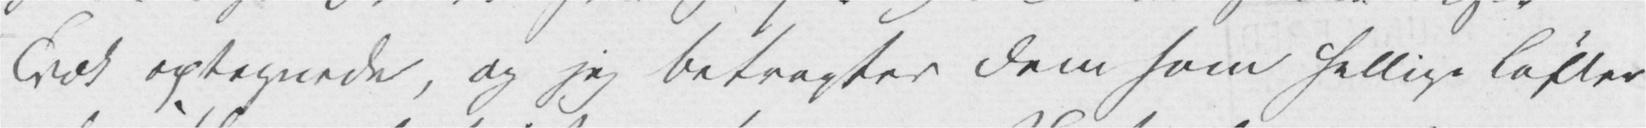Træk optegnede, og jeg betragter dem som hellige Løfter
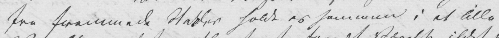tre fremmede Stakler holde os sammen i et lille
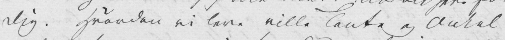Dig. Hvordan vi leve ville Tante og Onkel
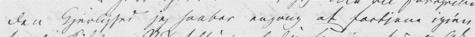den Kjerlighed jeg haaber engang at fortjene igjen
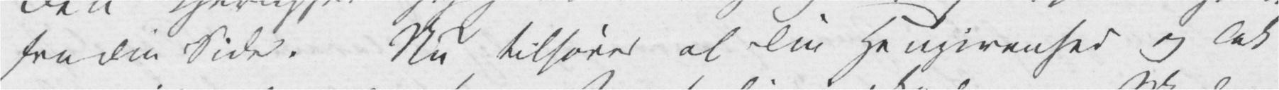fra Din Side. Nu tilhører al Din Hengivenhed og Tak-
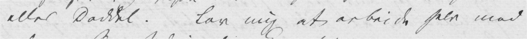eller Daddel. Lov mig at arbeide selv mod
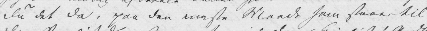Du det da, paa den eneste Maade som staaer til
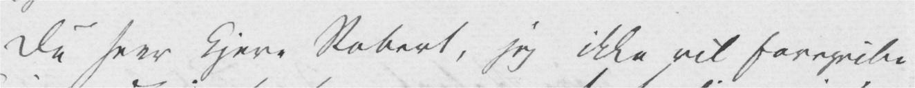Du seer kjere Robert, jeg ikke vil foregribe
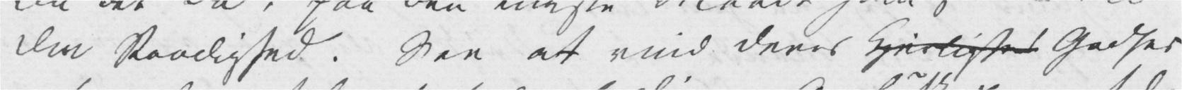Din Raadighed. See at vinde deres Kjerlighed Godhed,
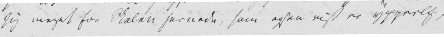sig meget for Skolen hernede, som ogsaa vist er ypperlig,
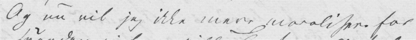Og nu vil jeg ikke mere moralisere for
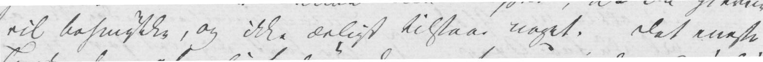vil besmykke, og ikke ærligt tilstaa noget. Det eneste
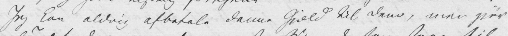Jeg kan aldrig afbetale denne Gjæld til dem, men gjør
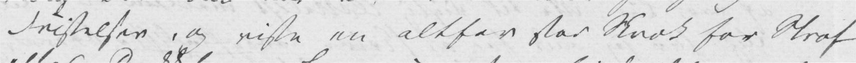Fristelser, og viste en altfor stor Skræk for Straf
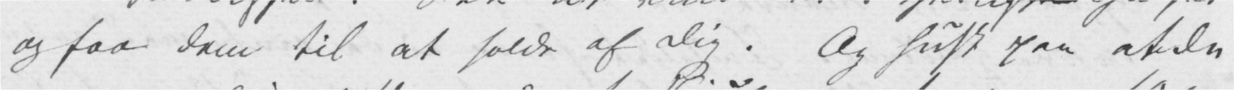og faa dem til at holde af Dig. Og husk paa at Du
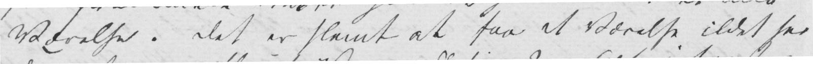Værelse. Det er slemt at faa et Værelse ildet her
